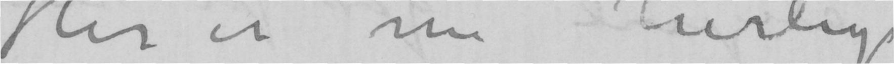Her er nu herlige
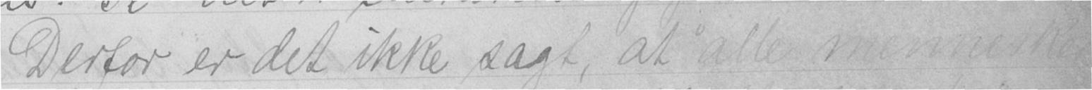Derfor er det ikke sagt, at "alle mennesker
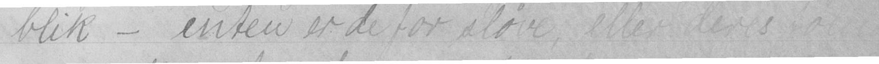blik - enten er de for sløve, eller deres tø
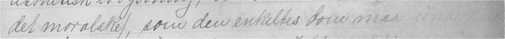det moralske), som den enkeltes dom maa underkaste
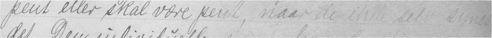pent eller skal være pent, naar de ikke selv synes
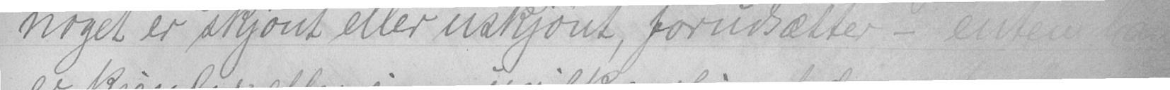noget er skjønt eller uskjønt, forudsætter - enten han
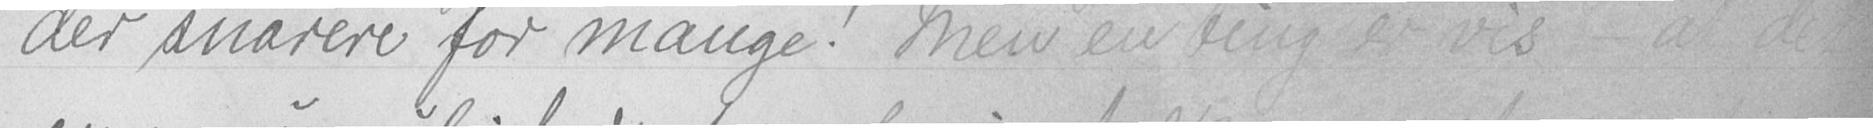der snarere for mange! Men en ting er vis - at der
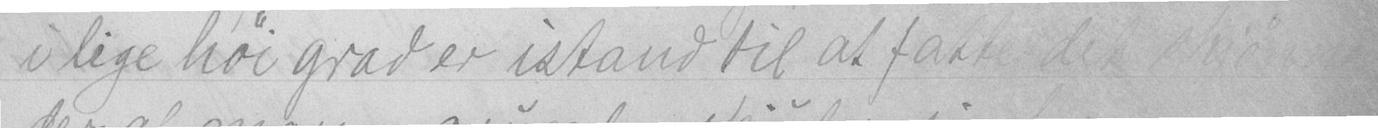i lige høi grad er istand til at fatte det skjønne
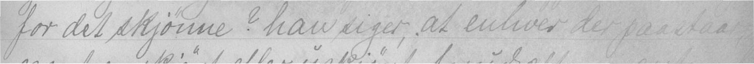for det skjønne? han siger, at enhver der paastaar
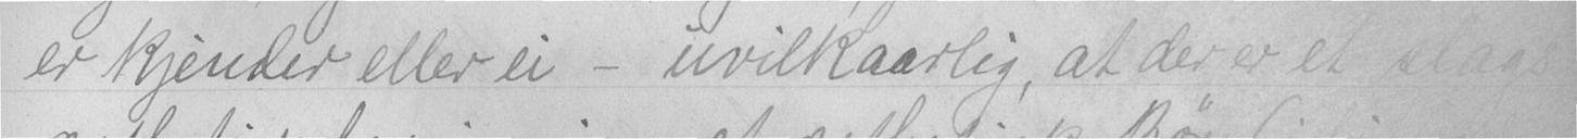erkjender eller ei - uvilkaarlig, at der er et slags
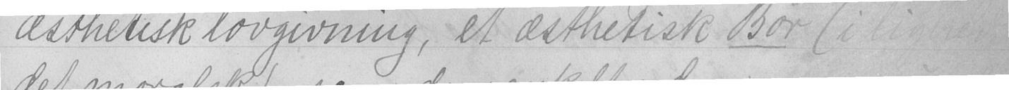æsthetisk lovgivning, et æsthetisk Bør (i lig
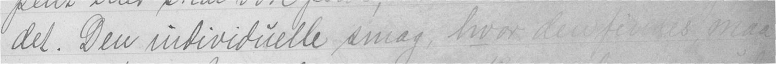det. Den individuelle smag, hvor den findes maa
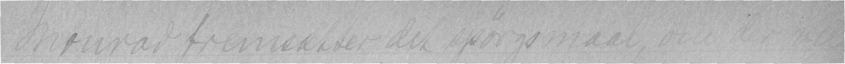Monrad fremsætter det spørgsmaal om der vel
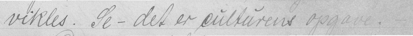vikles. Se - det er culturens opgave. -
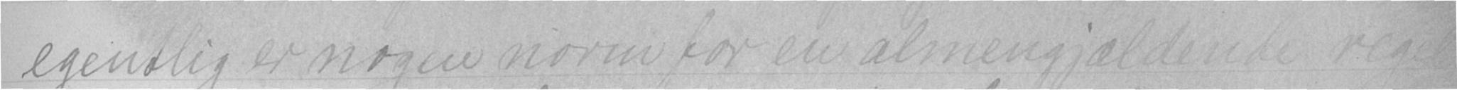egentlig er nogen norm for en almengjældende regel
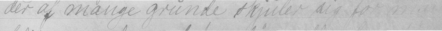der af mange grunde skjuler sig for m
